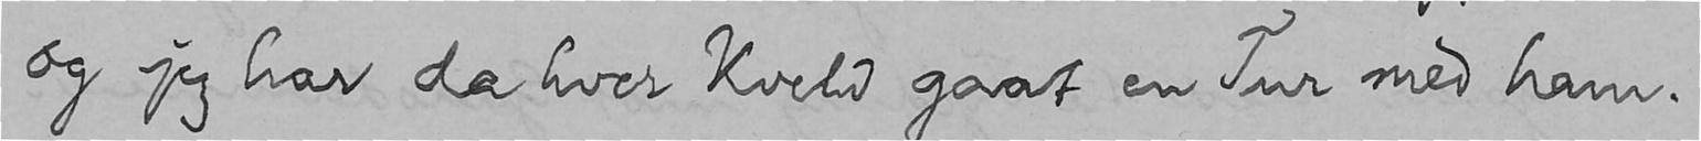og jeg har da hver Kveld gaat en Tur med ham.
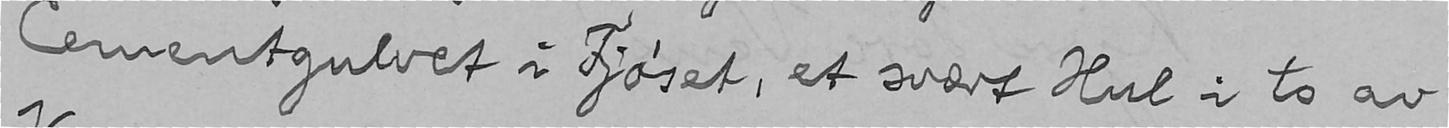Cementgulvet i Fjøset, et svært Hul i to av
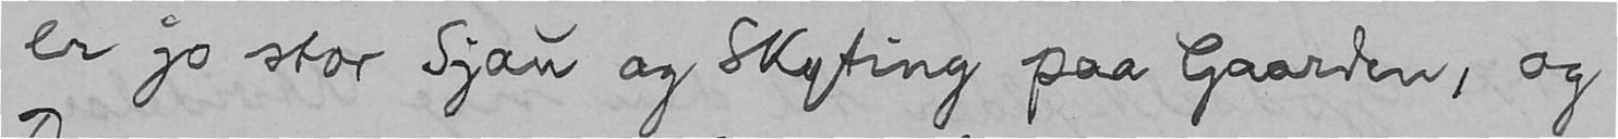er jo stor Sjau og Skyting paa Gaarden, og
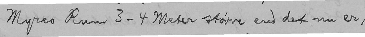Myres Rum 3-4 Meter større end det nu er,
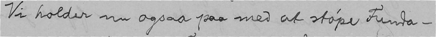Vi holder nu ogsaa paa med at støpe Funda-
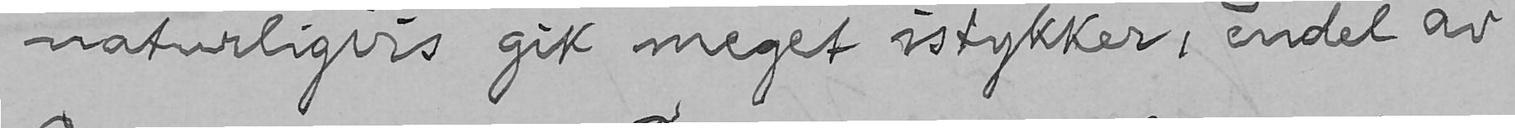naturligvis gik meget istykker, endel av
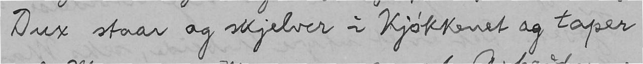Dux staar og skjelver i Kjøkkenet og taper
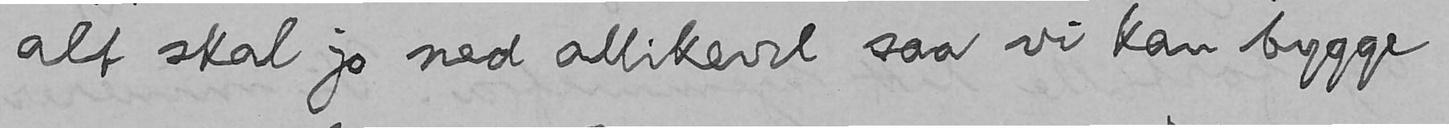alt skal jo ned allikevel saa vi kan bygge
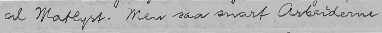al Matlyst. Men saa snart Arbeiderne
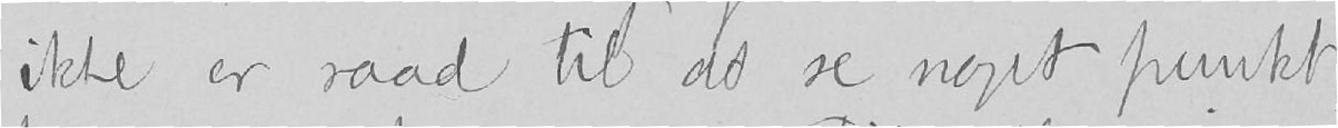ikke er raad til at se noget punkt,
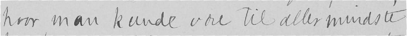hvor man kunde være til allermindste
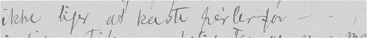ikke liger at kaste perler for – –,
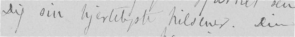Dig sin hjerteligste hilsener. Din
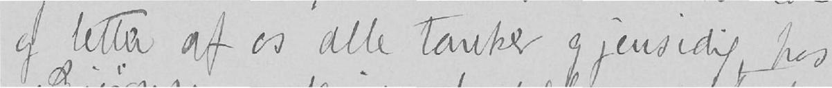og letter af os alle tanker gjensidig, hos
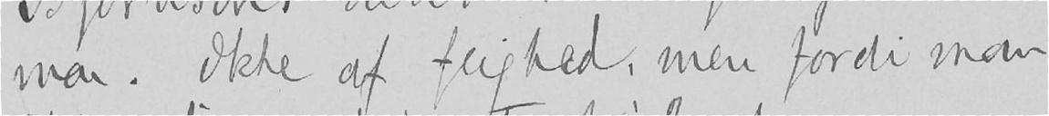man. Ikke af feighed, men fordi man
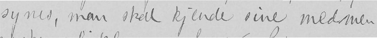synes, man skal kjende sine medmen
